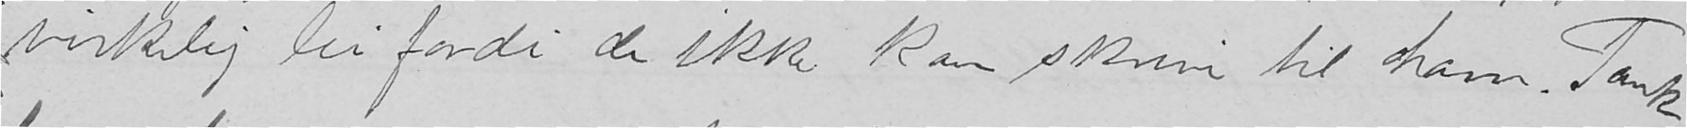virkelig lei fordi de ikke kan skrive til ham. Tænk
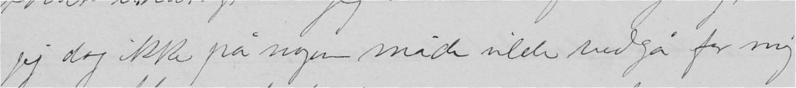jeg dog ikke på nogen måde vilde vedgå for mig
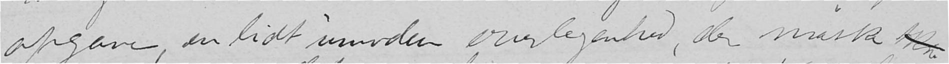opgave, en lidt umoden overlegenhed, der måske
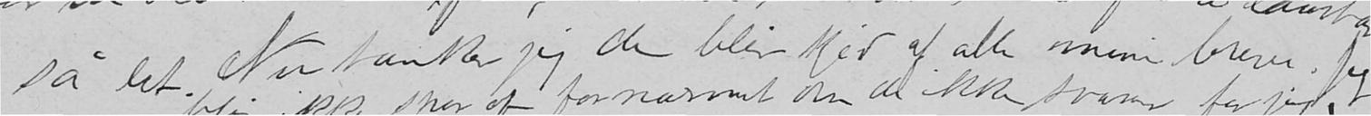så let. Nu tænker jeg de blir kjed af alle mine breve. Jeg
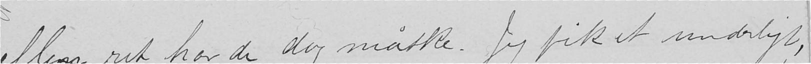Men ret har de dog måske. Jeg fik et underligt,
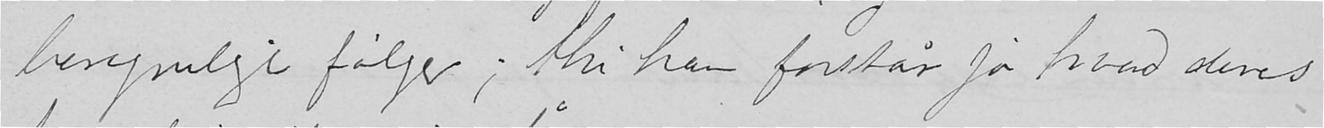beregnelige følger; thi han forstår jo hvad deres
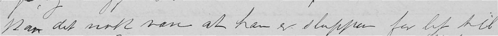kan det nok være at han er sluppen for let til
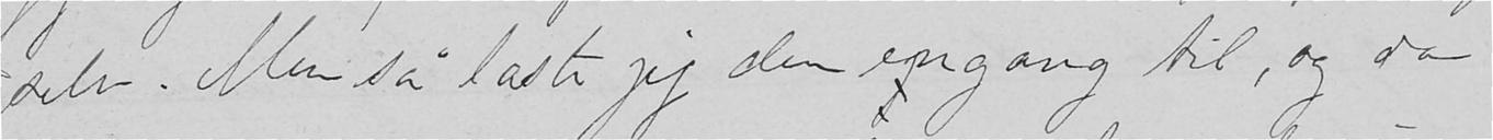selv. Men så læste jeg den engang til, og da
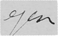egen
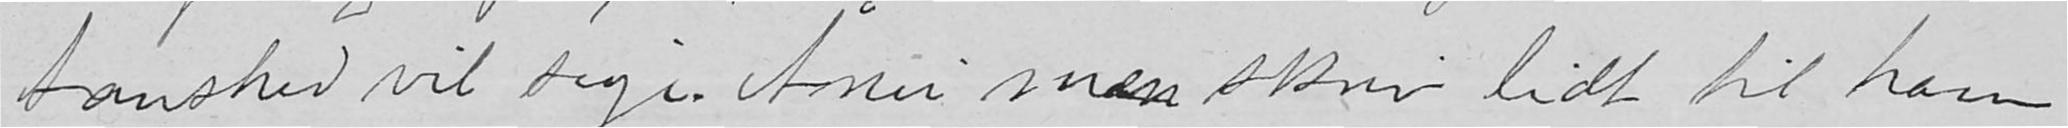taushed vil sige. Ånei men skriv lidt til ham
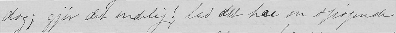dog; gjør det endelig! lad det hae en spørgende
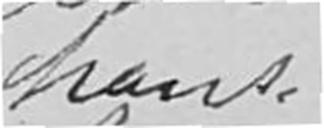hans
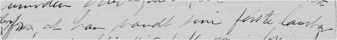fra, at han vandt sine første laurbær
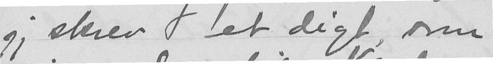jeg skrev et digt som
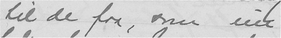til de faa, som nu
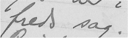freds sag.
